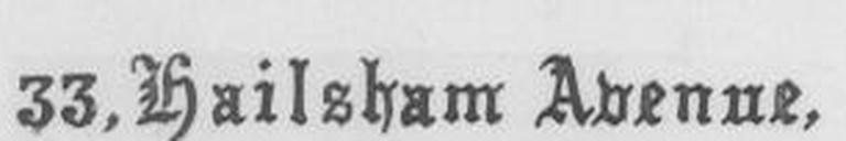33,Hailsham Adenue.
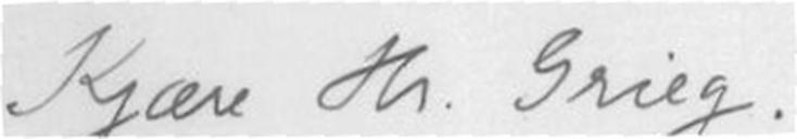Kjære Hr. Grieg.
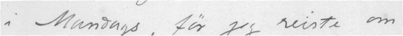i Mandags, før jeg reiste om
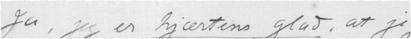Ja, jeg er hjærtens glad, at jeg
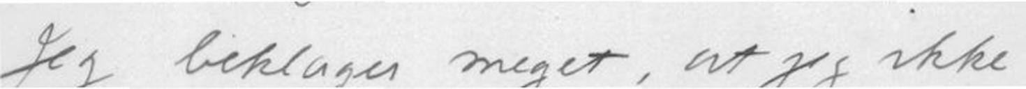Jeg beklager meget, at jeg ikke
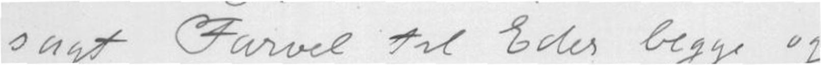sagt Farvel til Eder begge og
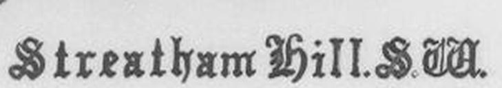Streatham Hill.S W.
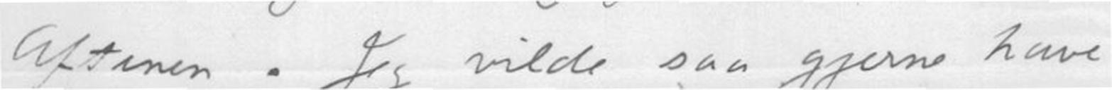Aftenen. Jeg vilde saa gjerne have
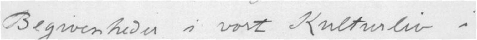Begivenheder i vort Kulturliv i
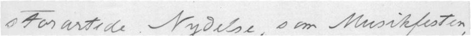den stoartede Nydelse, som Musikfesten,
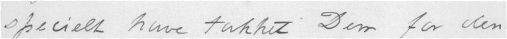specielt have takket Dem for
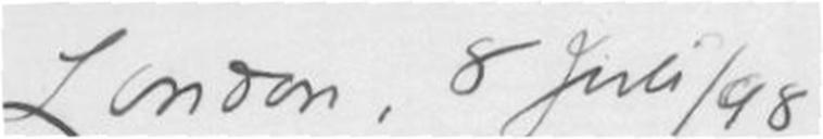London, 8 Juli/98
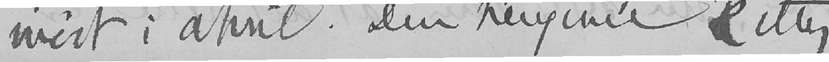mødt i april. Din hengivne Kitty.
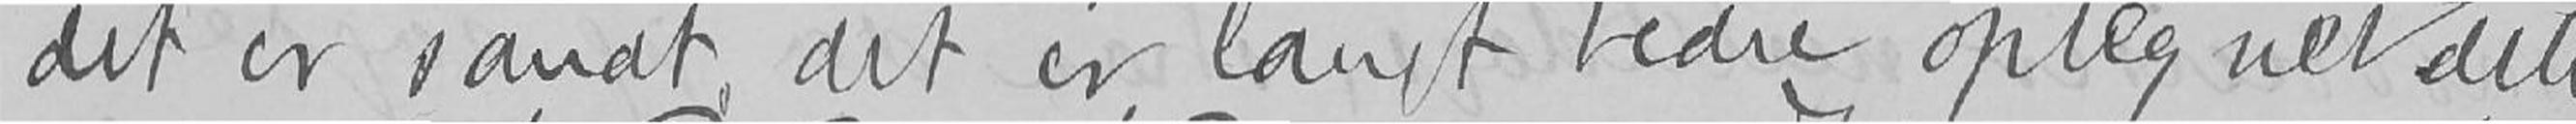det er sandt, det er langt bedre optegnet dette
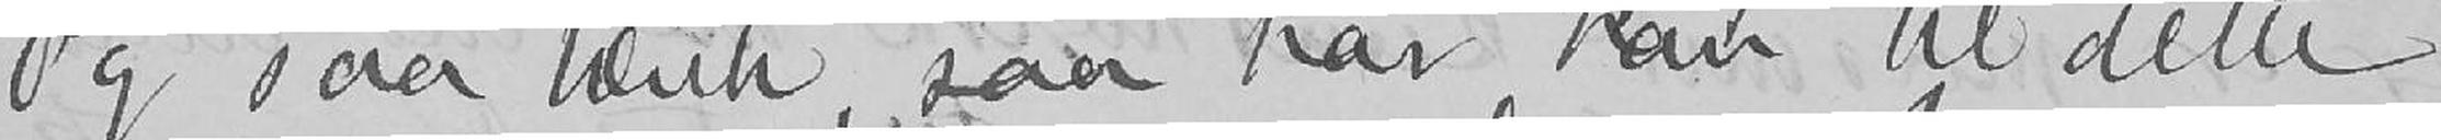Og saa tænk, saa har han til dette
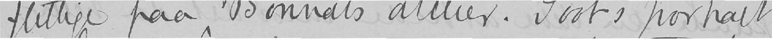flittige paa Bonnats atélier. Soots portrait
